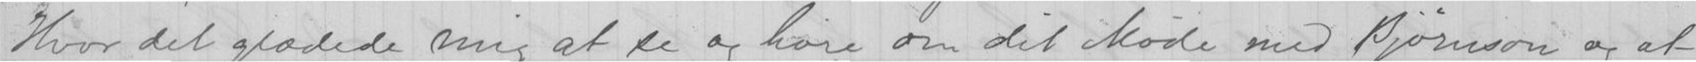Hvor det glædede mig at se og høre om det Møde med Bjørnson og at
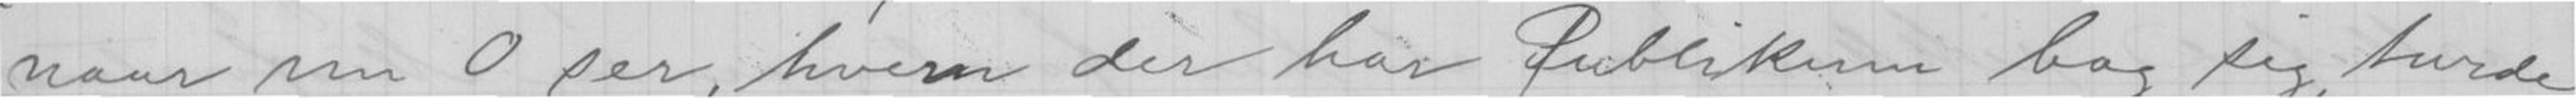naar nu O ser, hvem der har Publikum bag sig, turde
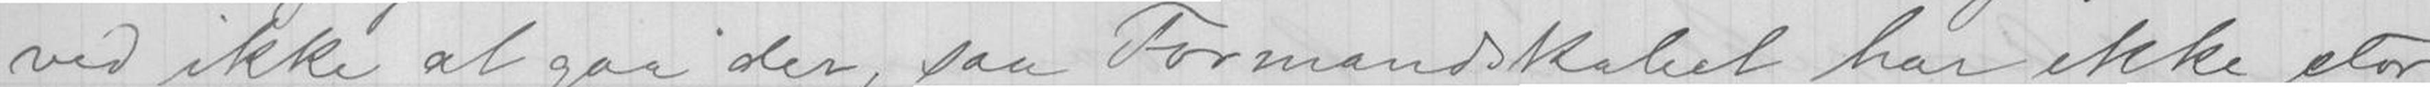ved ikke at gaa der, saa Formandskabet har ikke stor
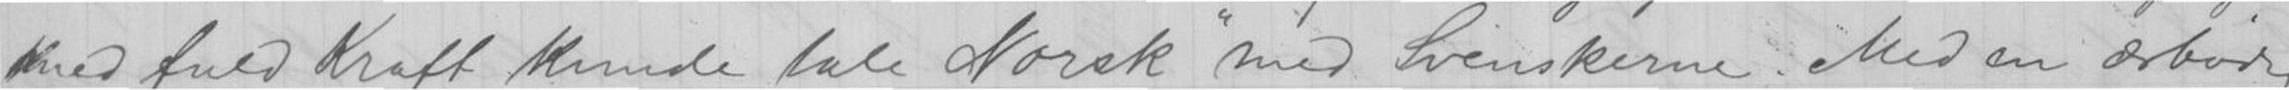med fuld Kraft kunde tale Norsk med Svenskerne. Med en ærbødig
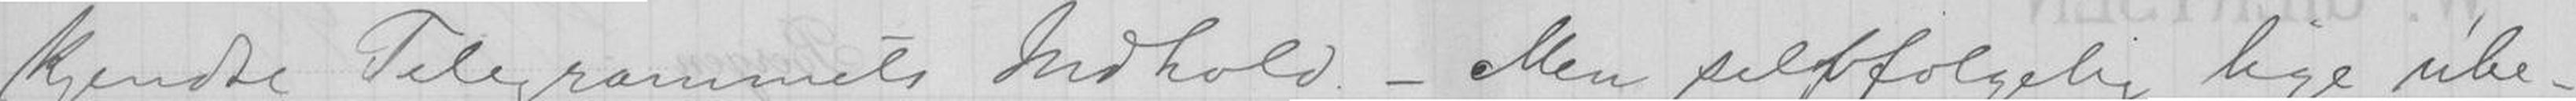kjendte Telegrammets Indhold. - Men selvfølgelig lige ube-
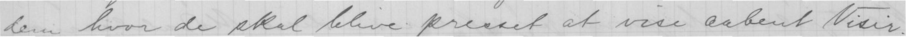dem, hvor de skal blive presset at vise aabent Visir;
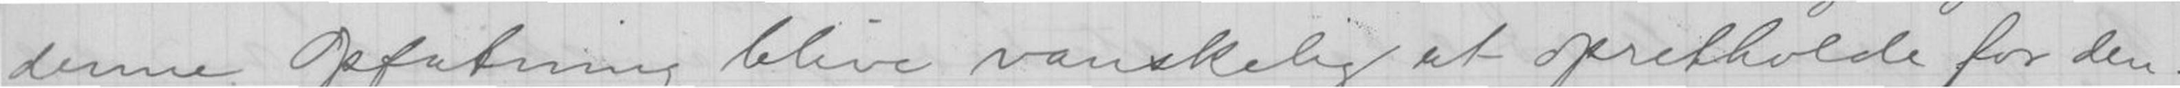denne Opfatning blive vanskelig at opretholde for den.
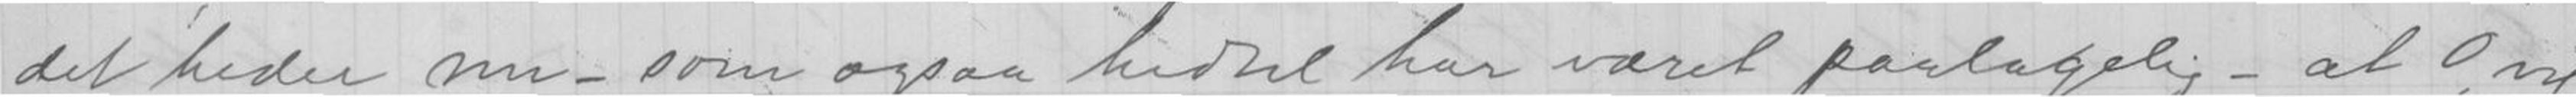det heder nu - som ogsaa hidtil har været paatagelig - at O, vil
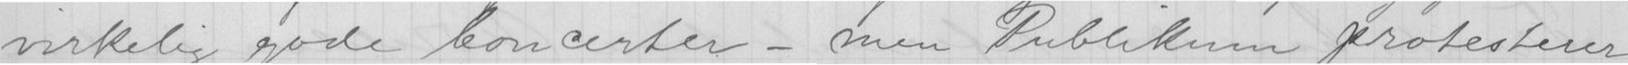virkelig gode Concerter - men Publikum protesterer
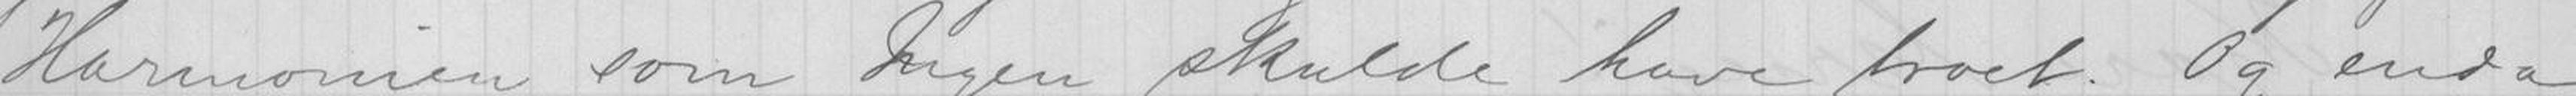Harmonien som Ingen skulde have troet. Og enda
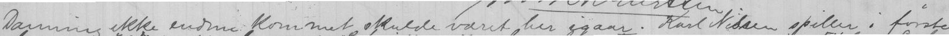Danning ikke endnu kommet skulde været her igaar. Karl Nissen spiller i første
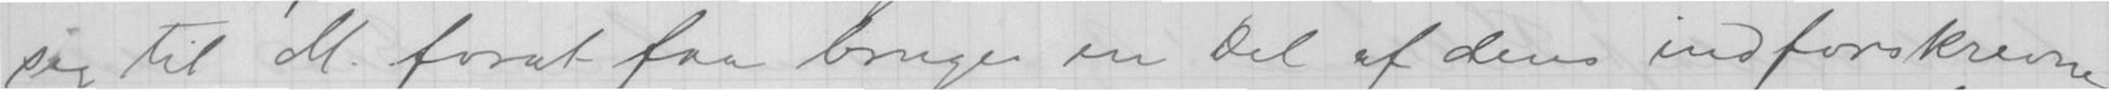sig til M. forat faa bruge en Del af dens indforskrevne
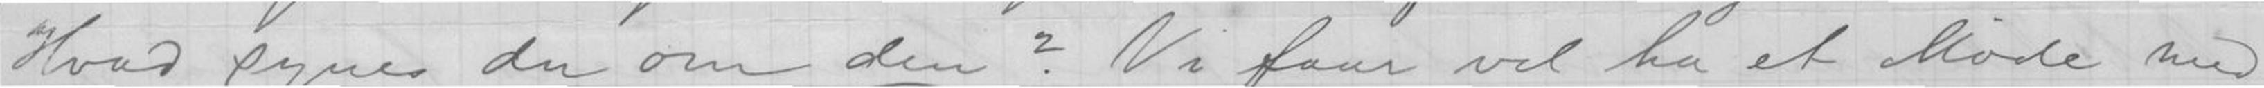Hvad synes du om den? Vi faar vel ha et Møde med
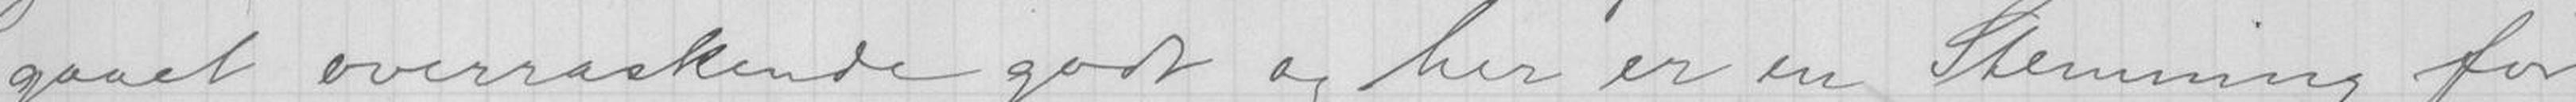gaaet overraskende godt og her er en Stemning for
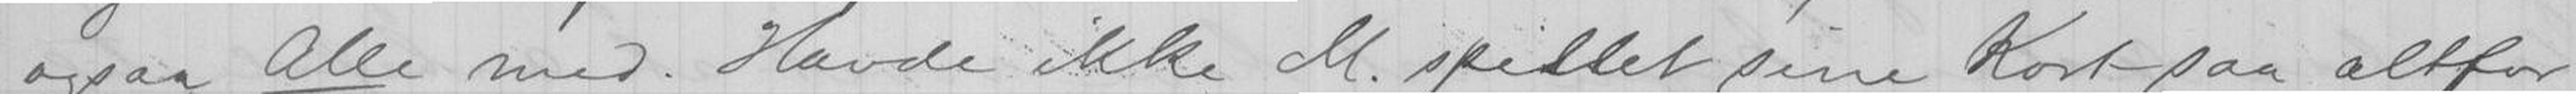ogsaa Alle med. Havde ikke M. spillet sine Kort saa altfor
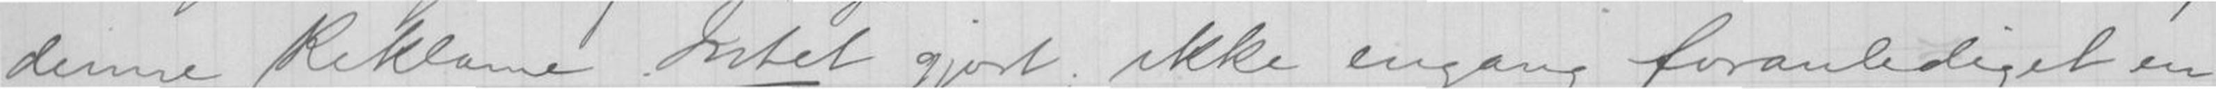denne Reklame Intet gjort; ikke engang foranlediget en
